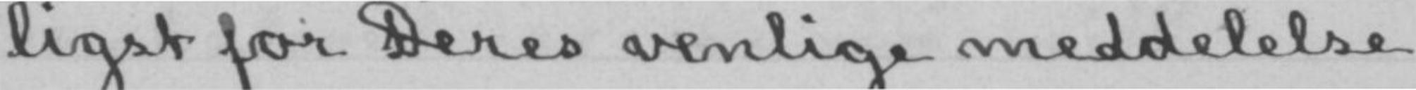ligst for Deres venlige meddelelse
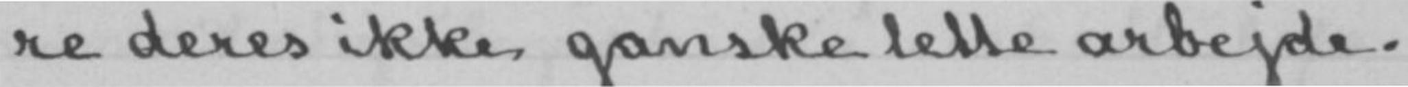re deres ikke ganske lette arbejde.
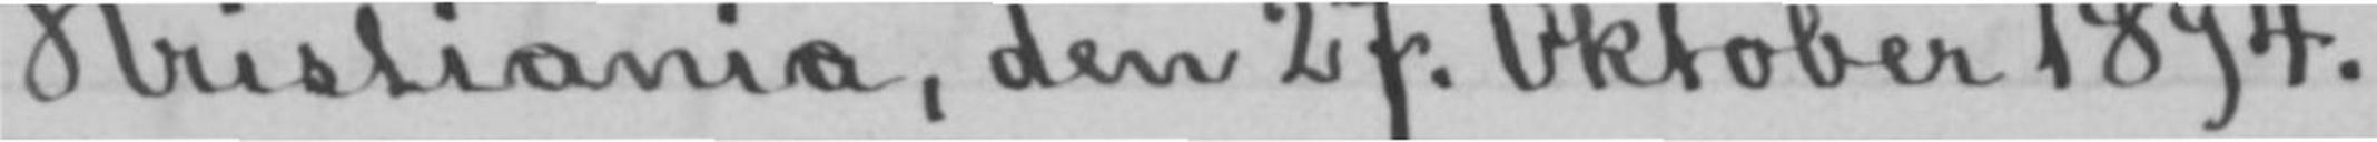Kristiania, den 27. Oktober 1894.
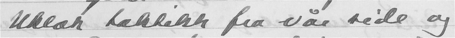Uklar taktikk fra vår side og
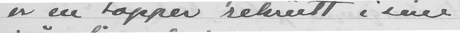er en tapper rekrutt i sine
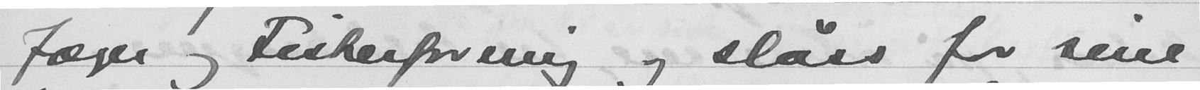Jæger og Fiskerforening og og slåss for sine
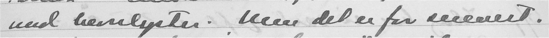med barnligheter. Men det er for snevert.
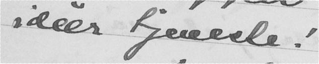ideers tjeneste!
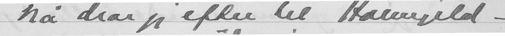Nå drar jeg efter til Holmgild -
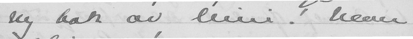ny bok av Nini. Men
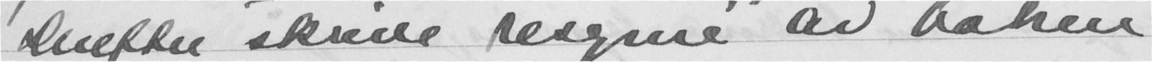Derefter skrive resgene av boken
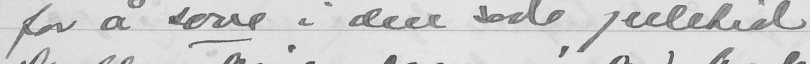for å leve i den søde juletid.
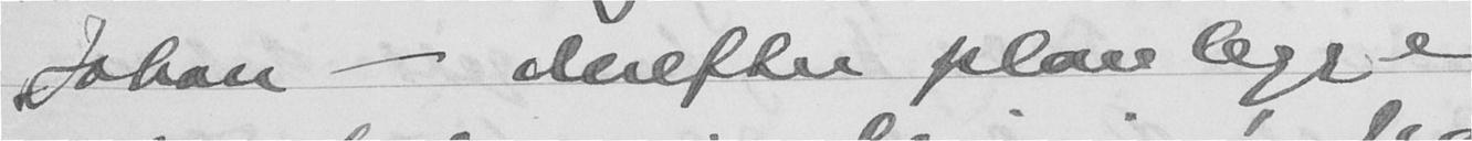Johan - derefter planlegge
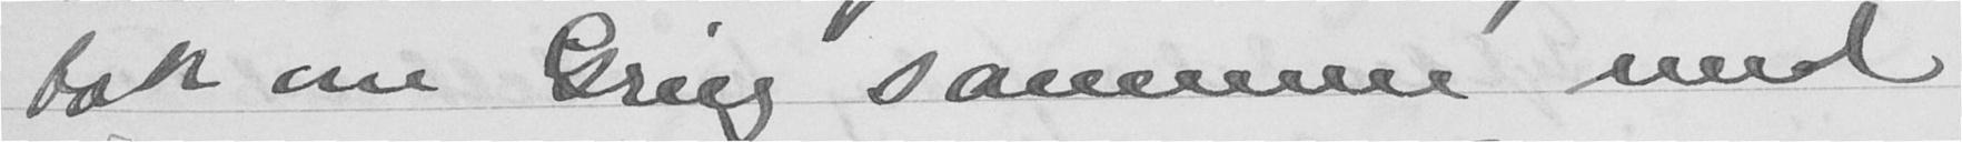bok om Grieg sammen med
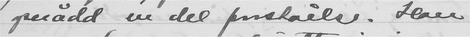opnådd en del forståelse. Han
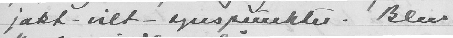jakt-vilt-synspunkter. Blev
